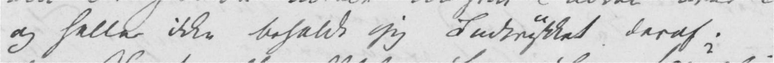og til at indbilde os at Verden er bedre og lykkeligere end den er. Der er en ældre Dame – gammel
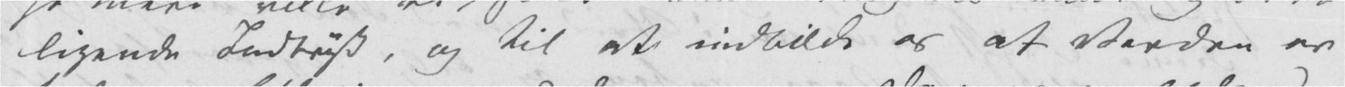trænger hun til den Selvskuffelse at alting er godt og vel. Jeg kunde nævne Dem flere der ikke have mægtet Indtrykket af disse
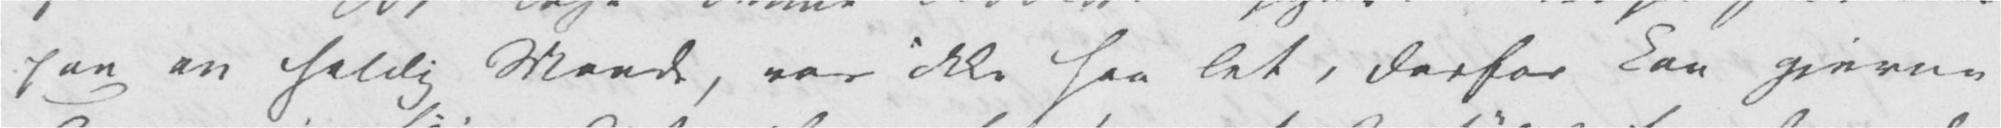paa en heldig Maade, var ikke saa let, derfor kan gjerne
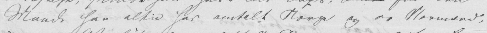Maade han altid har omtalt Norge og os Normænd,
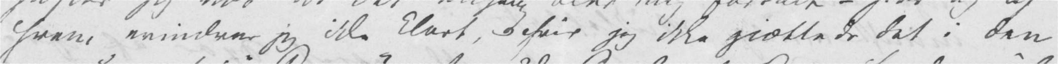hvem erindrer jeg ikke klart, hvis jeg ikke gjættede det i den
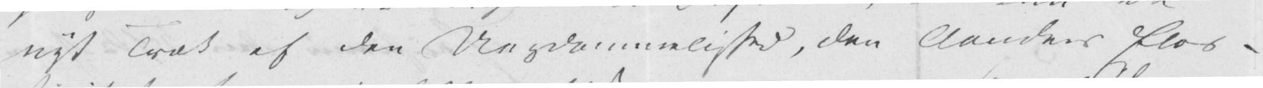nyt Træk af den Ungdommelighed, den Aandens
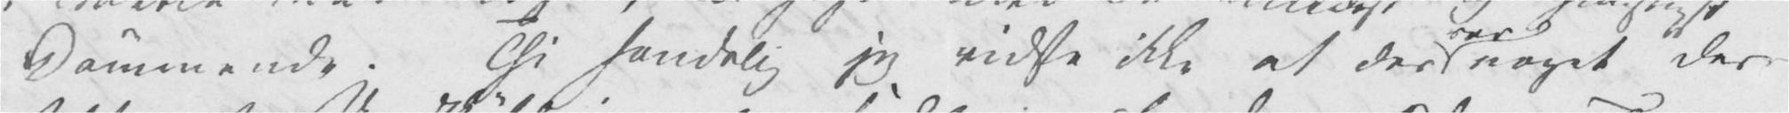Dømmende. Thi sandelig jeg vidste ikke at der var noget der
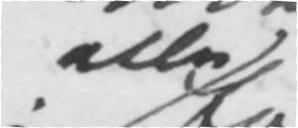beskuer Livet
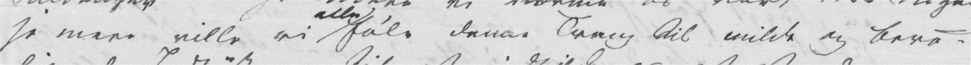paa Overfladen, netop frit for Overdrivelse, saa er det gaaet hende accurat som Professoren, hun har knurret over det sørgelige, saa inderlig
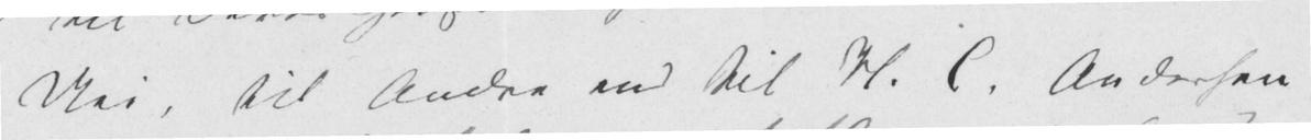Nei, til Andre end til H. C. Andersen
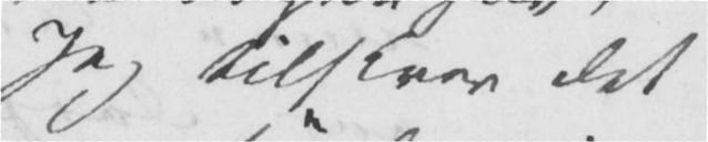milde og beroligende Indtryk,
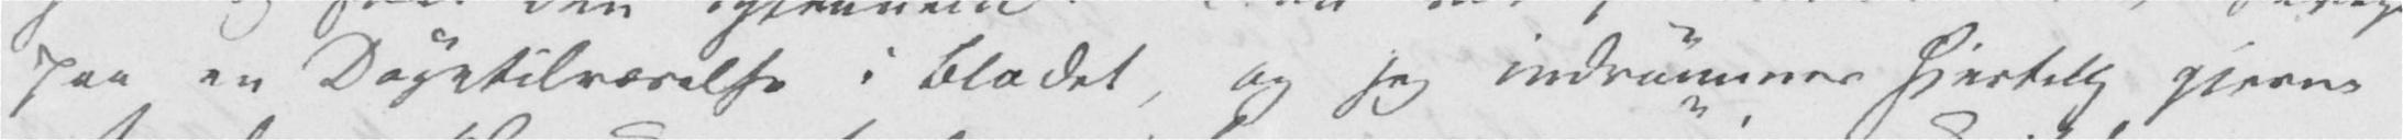paa en Døgntilværelse i Bladet, og jeg indrømmer hjertelig gjerne
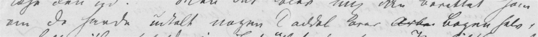have for sørgelige og sønderrivende Skildringer. Jo mere vi nærme os vort Livs Udgang jo mere ville vi alle føle denne Trang til
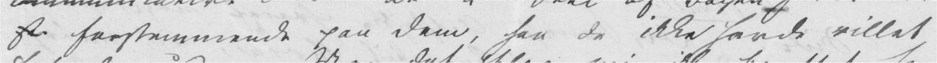st forstemmende paa Dem, saa De ikke havde villet læse den ud. Men det blev mig ikke berettet
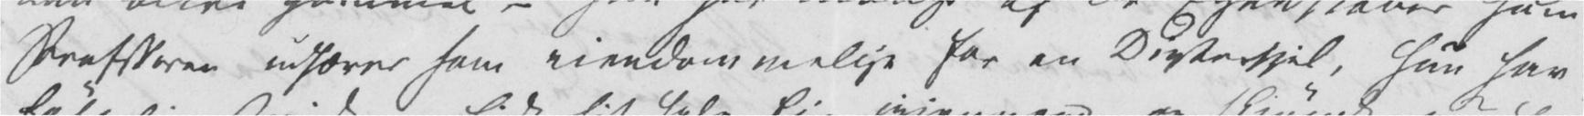Endnu engang Undskyldning for at dette kommer saa seent, jeg bliver ikke ganske rolig før jeg har seet Dem og da har
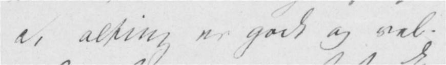Elasticitet
